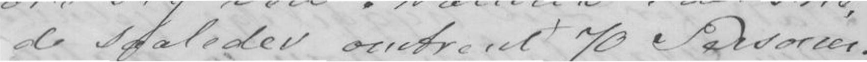de saaledes omtrent 70 Personer.
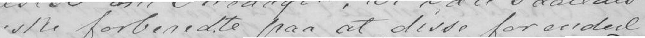ske forberedte paa at disse for endeel
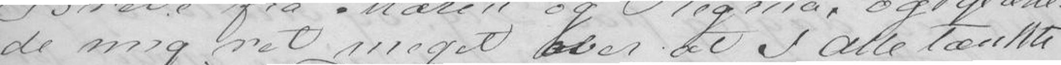de mig ret meget over at I Alle tænkte
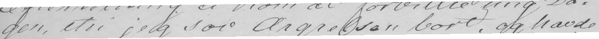gen, thi jeg sov Ærgrelsen bort, og havde
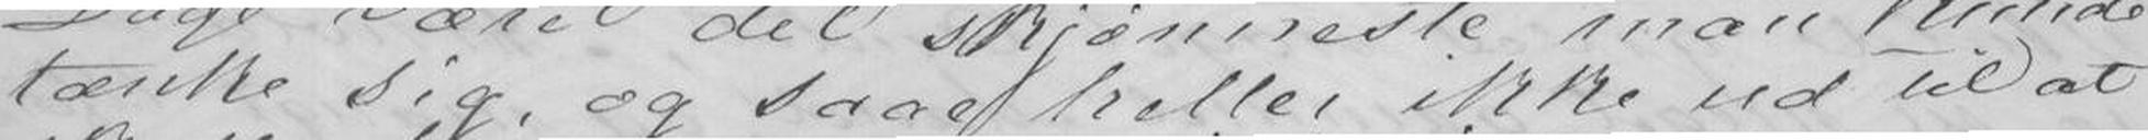tænke sig, og saae heller ikke ud til at
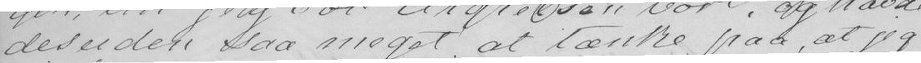desuden saa meget at tænke paa, at jeg
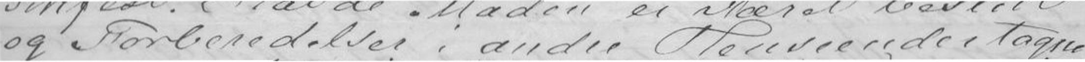og Forberedelser i andre Henseender tagne,
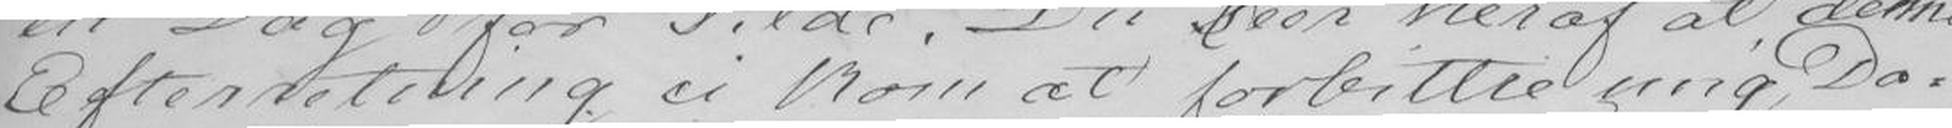Efterretning ei kom at forbittre mig Da-
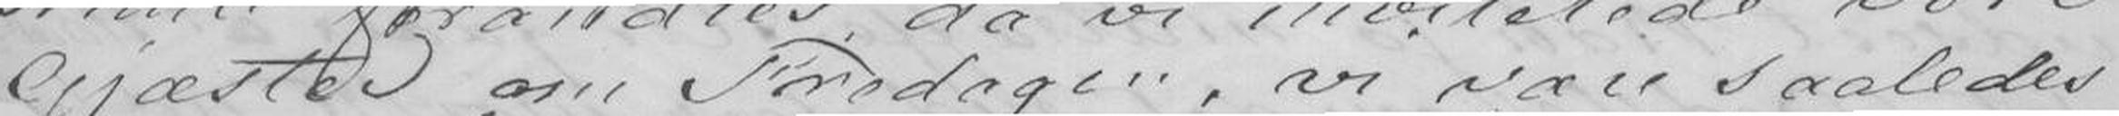Gjæster om Fredagen, vi være saaledes
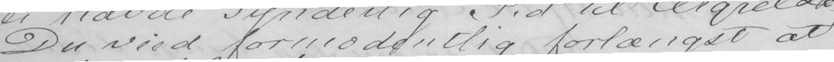Du veed formodentlig forlængst at
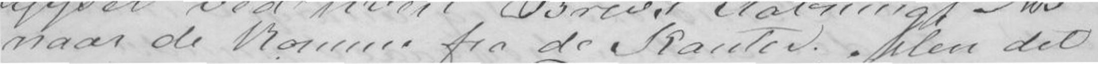naar de komme fra de Kanter. Men det
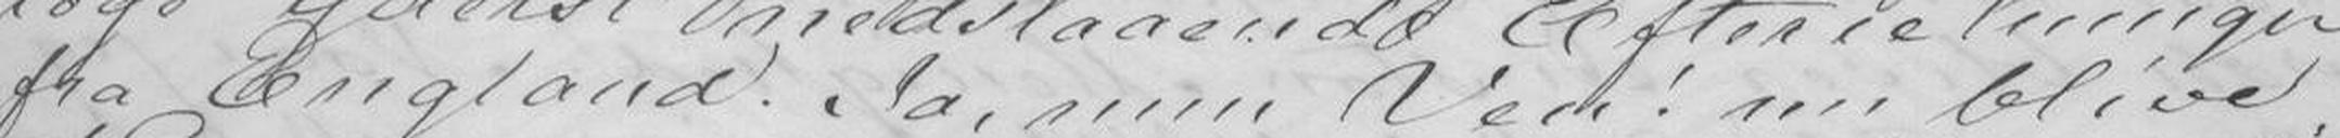fra England. Ja, min Ven! nu blive
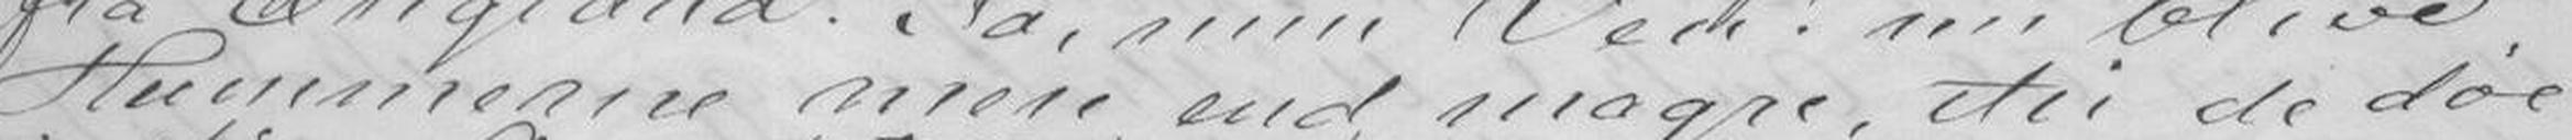Hummerne mere end magre, thi de døe
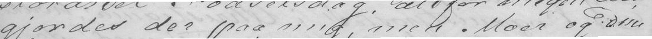gjordes der paa mig, men Moer og Dine
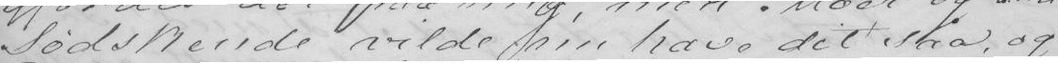Sødskende vilde nu have det saa, og
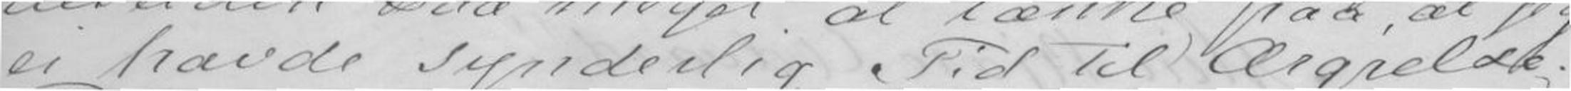ei havde synderlig Tid til Ærgrelse.
